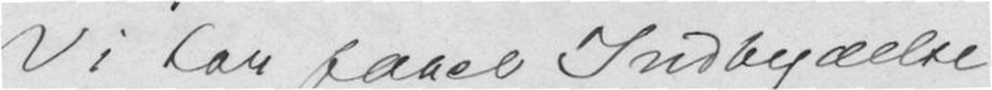Vi har faaet Indbydelse
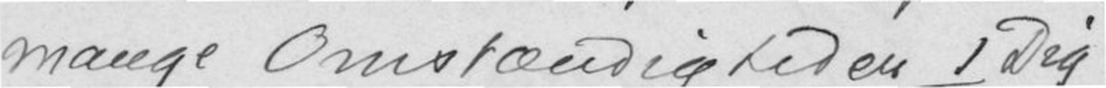mange Omstændigheden - Dig-
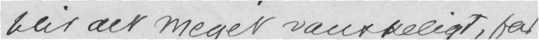blir det meget vanskeligt, for
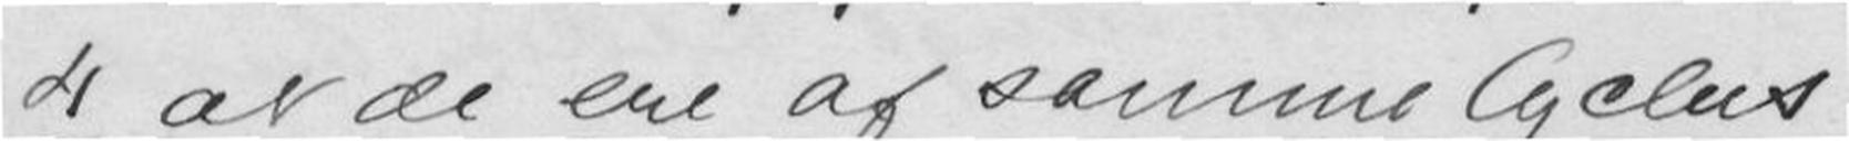4 at de ere af samme Cyclus
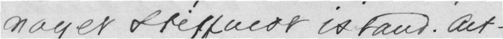noget istand. Alt-
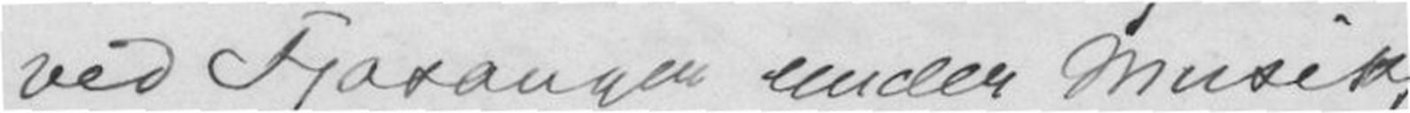ved Fjosangen inden Musik
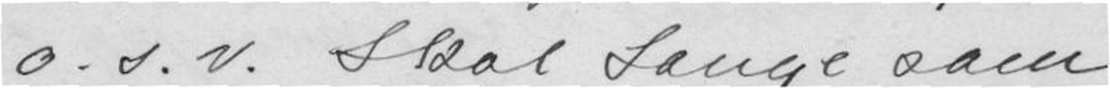o.s.v. Skal Sange som
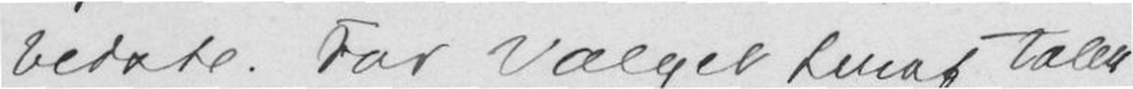betate. For valget deraf Taler
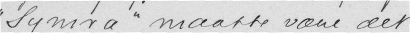"Symra" maatte være det
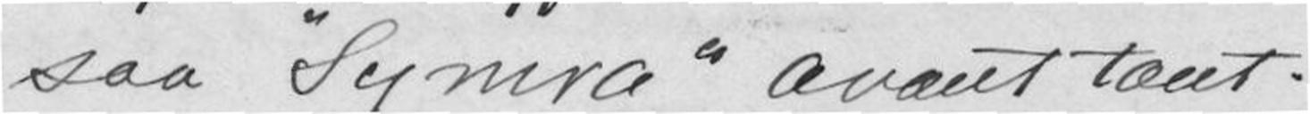saa "Symra" avænttænt.
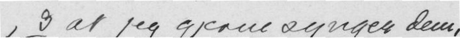,3 at jeg gjerne synger dem,
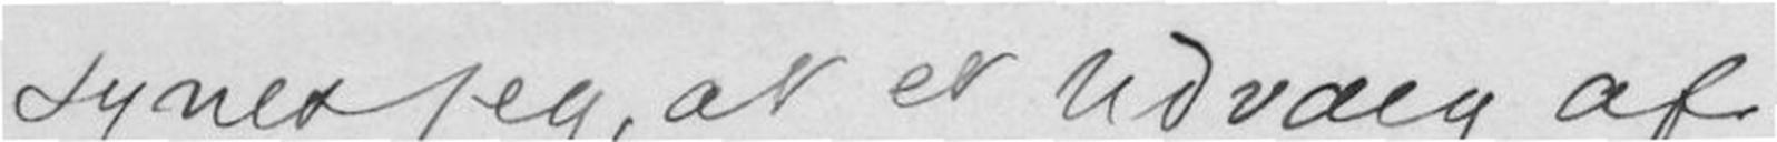synes jeg, at et udvalg af
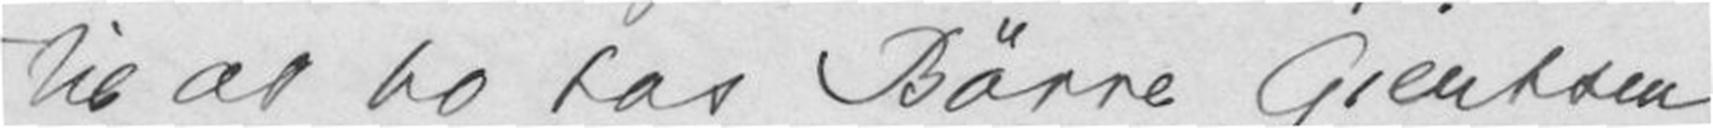til at bo hos Børre Giertsen
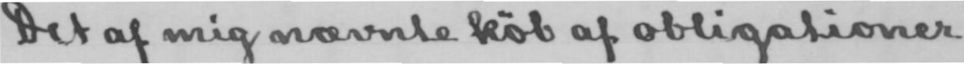Det af mig nævnte køb af obligationer
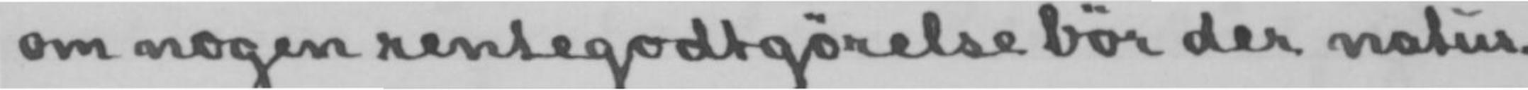om nogen rentegodtgørelse bør der natur-
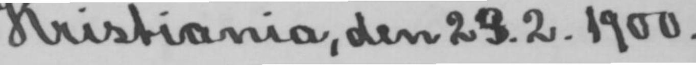Kristiania, den 23.2.1900.
